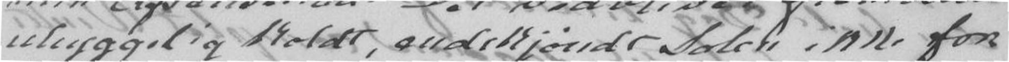uhyggelig koldt, endskjøndt Solen ikke for-
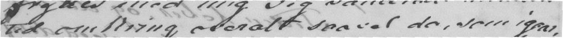tid omkring overalt saavel da, som igaar,
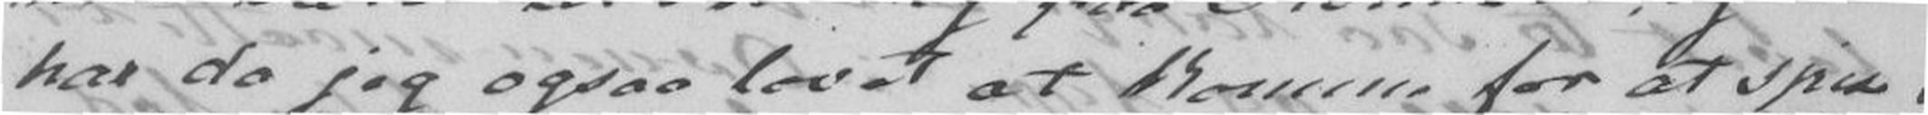har da jeg ogsaa lovet at komme for at spise
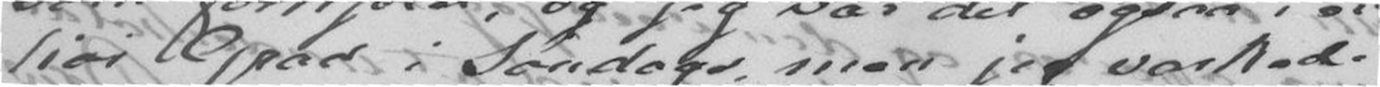høi Grad i Søndags, men jeg vaskede
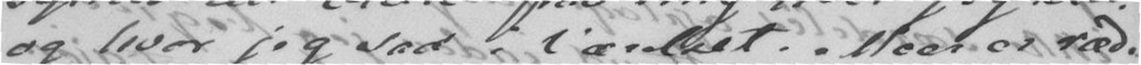og hvor jeg sad i Værelset. Moer er ræd-
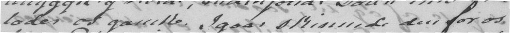lader os ganske. Igaar skinnede den for os,
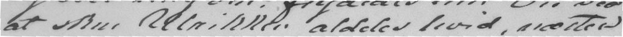at skue Ulrikken aldeles hvid, næsten
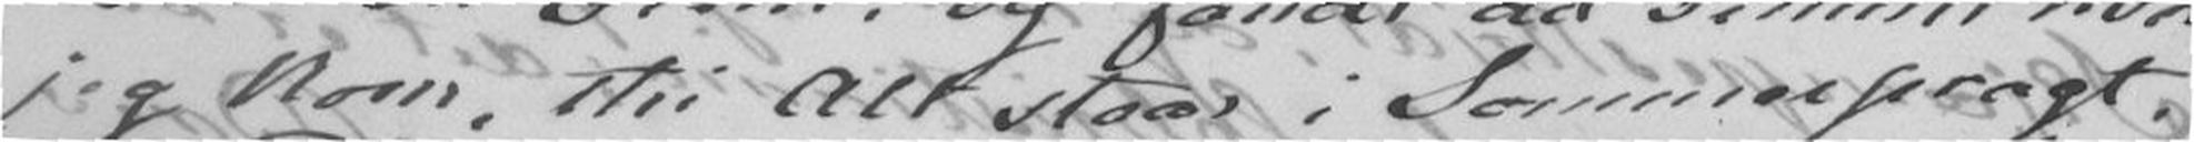jeg kom, thi Alt staar i Sommerpragt,
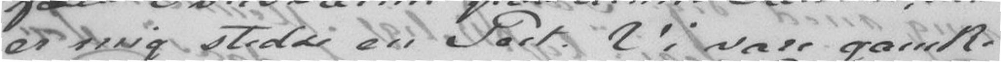er mig stedse en Part. Vi vare ganske
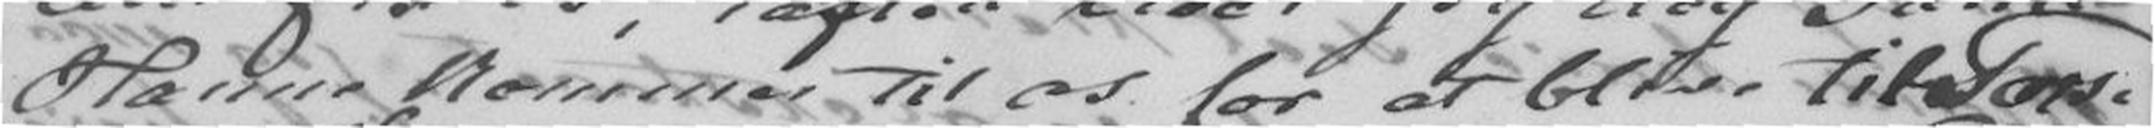Hanne kommer til os for at blive til Tors-
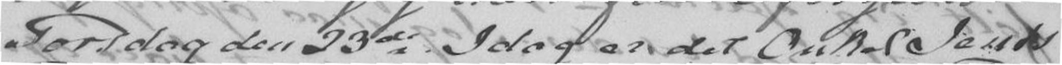Torsdag den 23de. Idag er det Onkel Jens
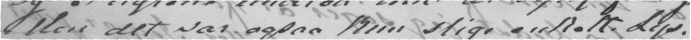Men det var ogsaa kun slige enkelte Lys-
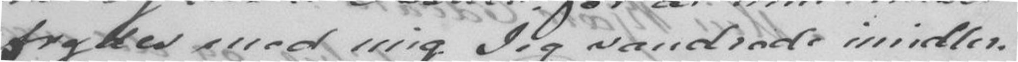frydes med mig Jeg vandrede imidler-
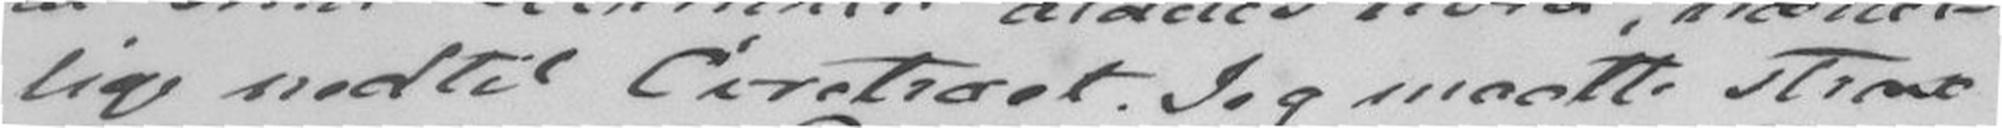lige nedtil Øvretræet. Jeg maatte strax
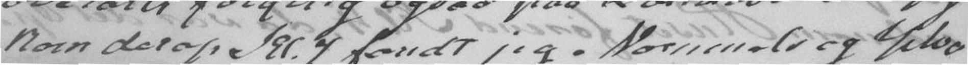kom derop Kl.7 fandt jeg Nommels og Yelva
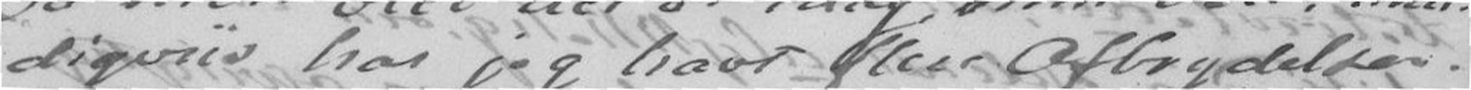digvis har jeg havt flere Afbrydelser.
